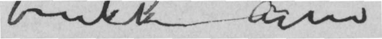brukken arm
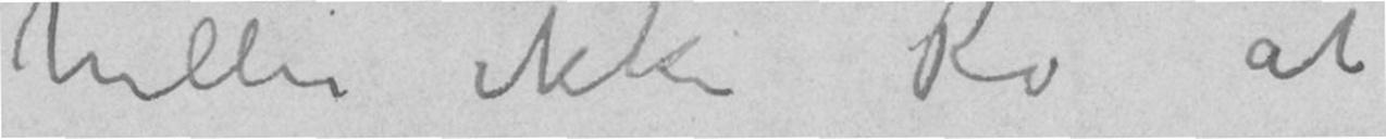heller ikke Ro at
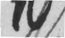10)
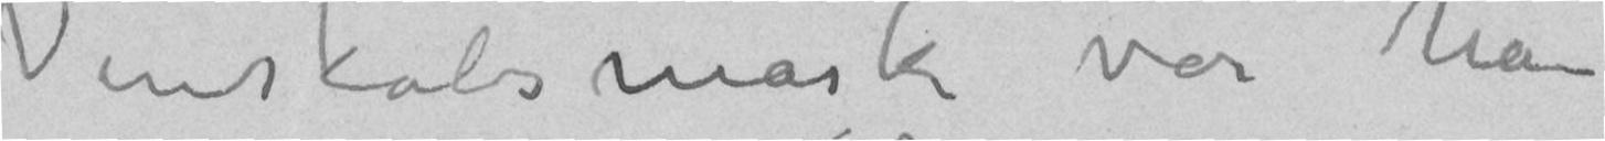Venskabsmaske var han
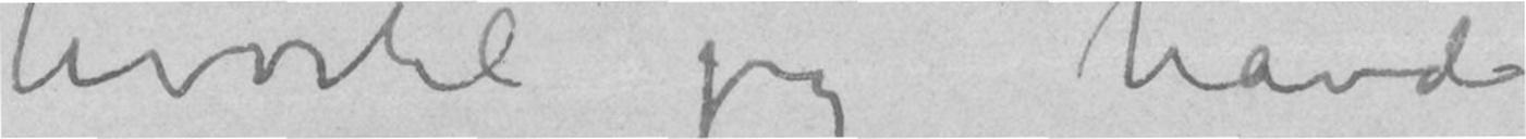hvortil jeg havde
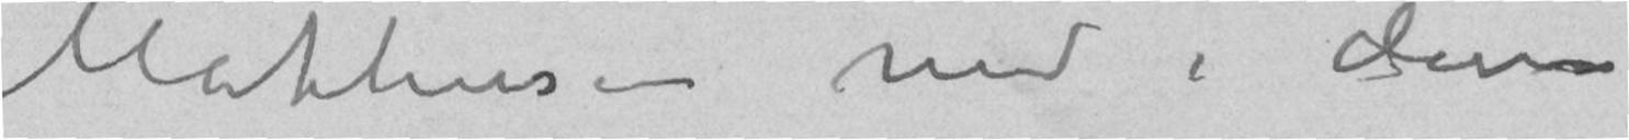Mathiesen med i den
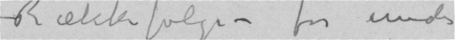–Rækkefølge – for under
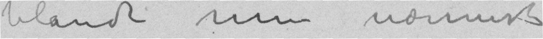blandt min nærmeste
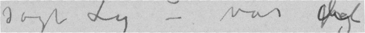søgt Ly – var jegdet
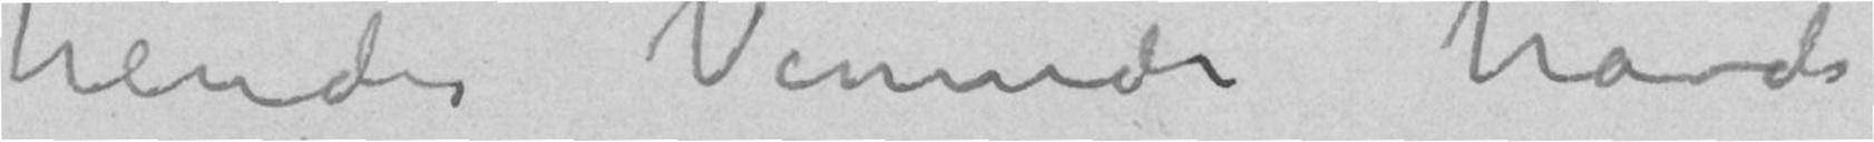hendes Veninde havde
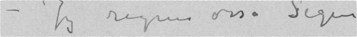– Jeg regner osså Sigur
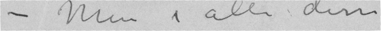Men i alle disse
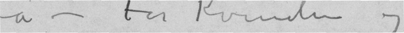få – For Kvinden og
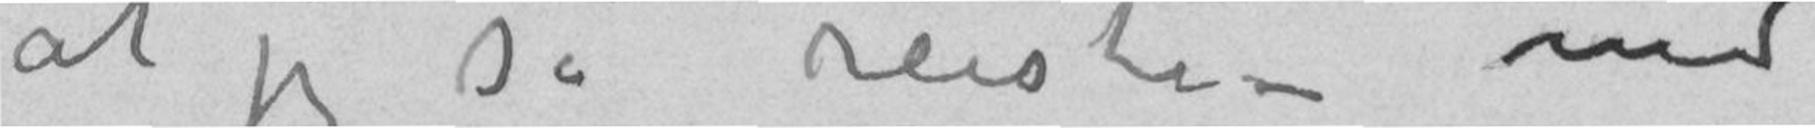at jeg så reiste med
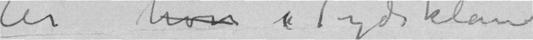År hvor i Tydskland
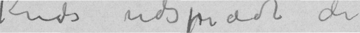Kreds udsprædt de
